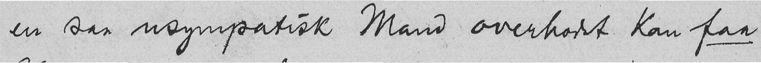en saa usympatisk Mand overhodet kan faa
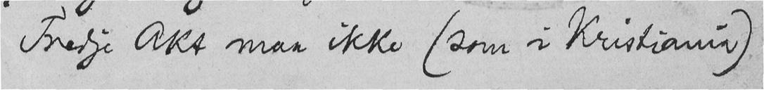Tredje Akt maa ikke (som i Kristiania)
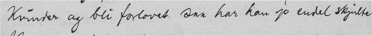Kvinder og bli forlovet saa har han jo endel skjulte
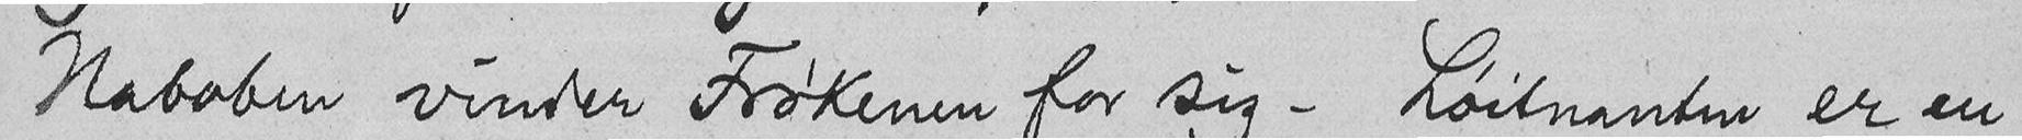Naboben vinder Frøkenen for sig. Løitnanten er en
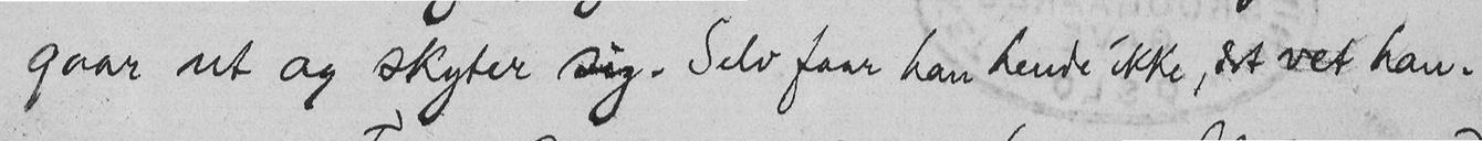gaar ut og skyter sig. Selv faar han hende ikke, det vet han.
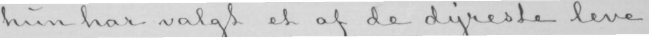hun har valgt et af de dyreste leve-
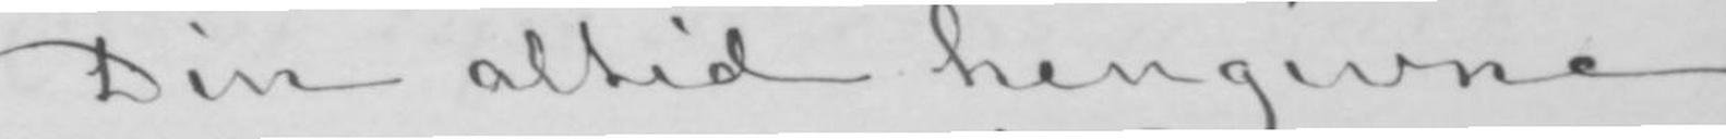Din altid hengivne
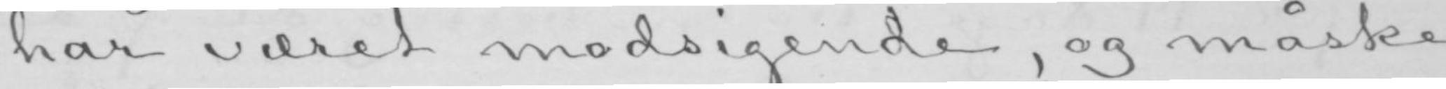har været modsigende, og måske
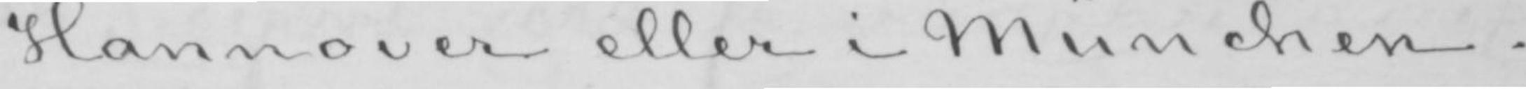Hannover eller i München.
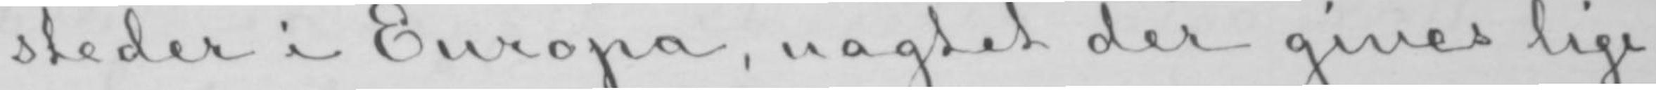steder i Europa, uagtet der gives lige-
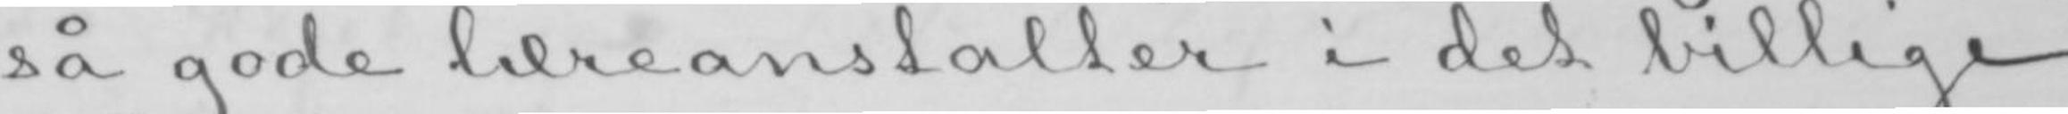så gode læreanstalter i det billige
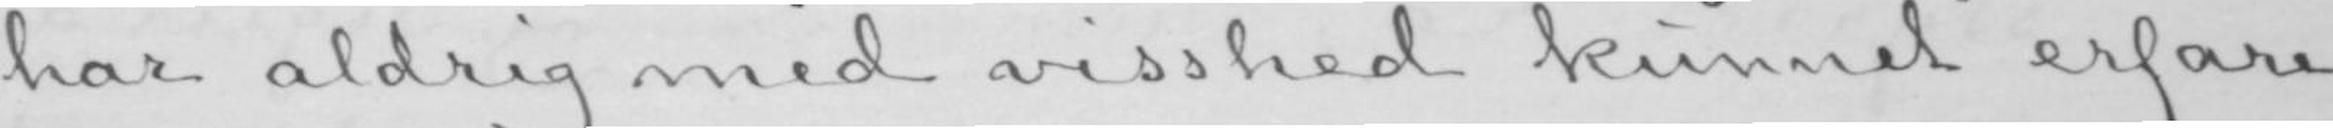har aldrig med visshed kunnet erfare
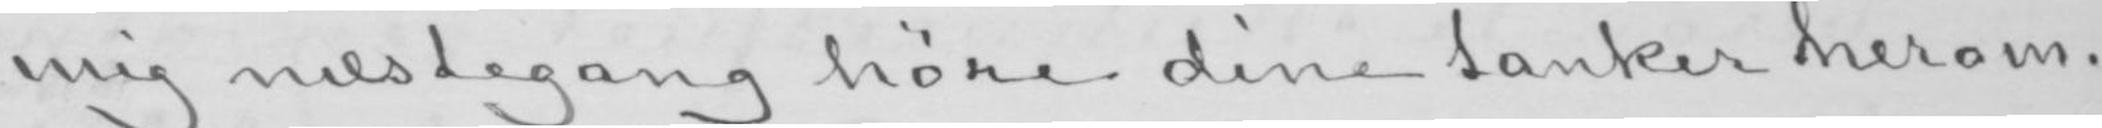mig næstegang høre dine tanker herom.
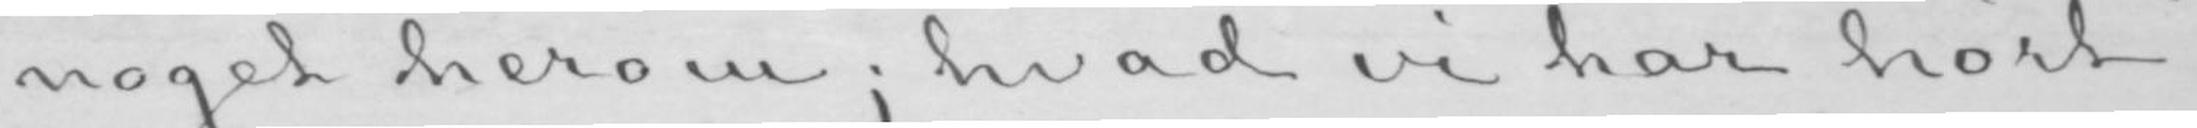noget herom; hvad vi har hørt,
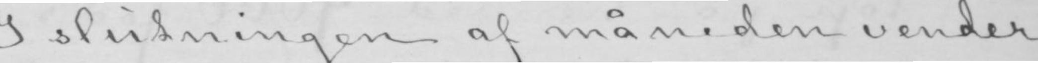I slutningen af måneden vender
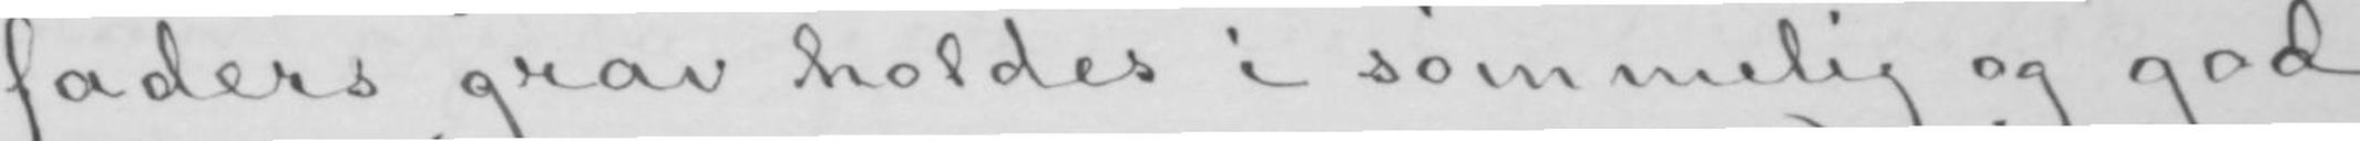faders grav holdes i sømmelig og god
